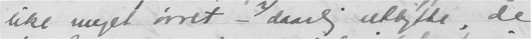like meget øiret - 2/ daarlig utbytte, de
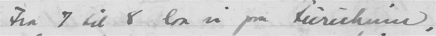Fra 7 til 8 laa vi paa Furuheim,
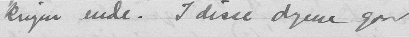krigen ende. I disse dagene gaar
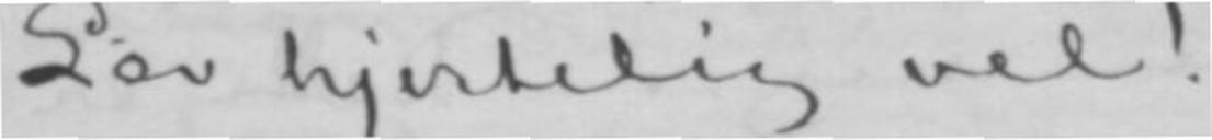Lev hjertelig vel!
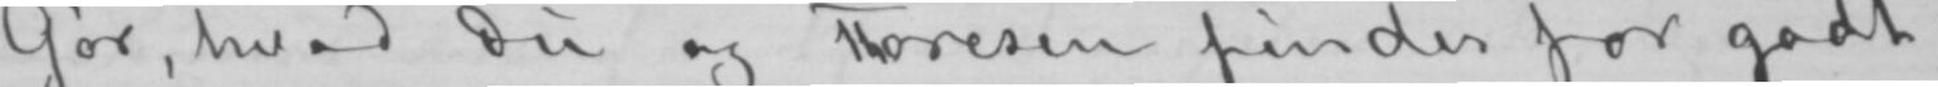Gør, hvad Du og Thoresen finder for godt;
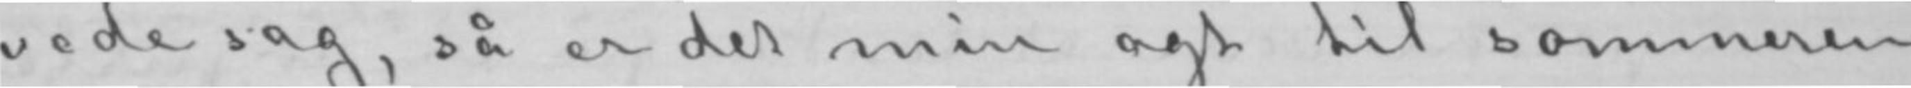vede sag, så er det min agt til sommeren
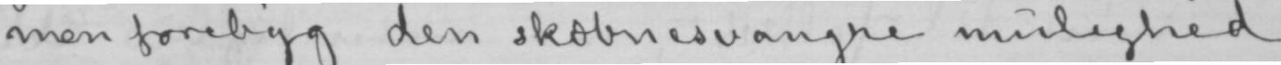men forebyg den skæbnesvangre mulighed
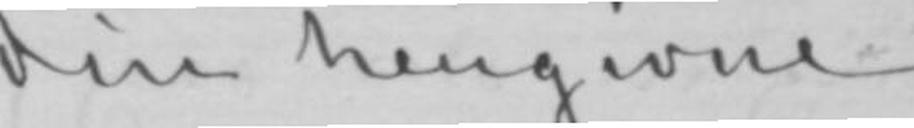Din hengivne
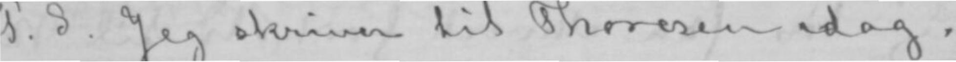P. S. Jeg skriver til Thoresen idag.
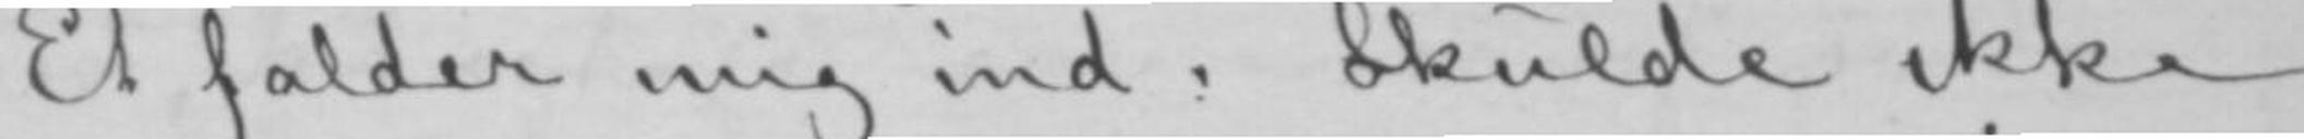Et falder mig ind. Skulde ikke
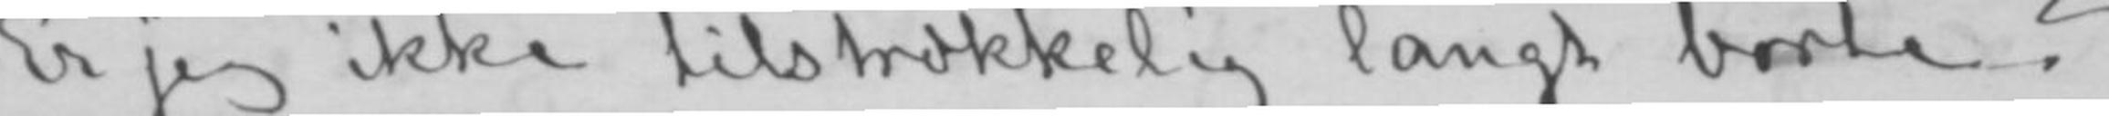Er jeg ikke tilstrækkelig langt borte?
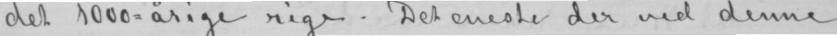det 1000-årige rige. Det eneste der ved denne
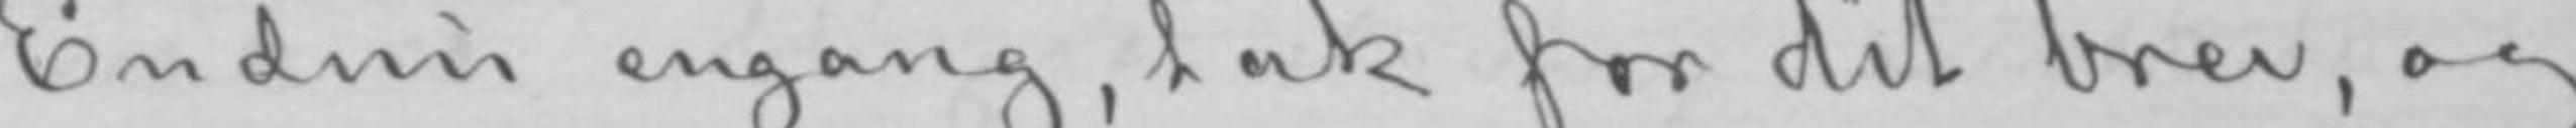Endnu engang, tak for dit brev, og
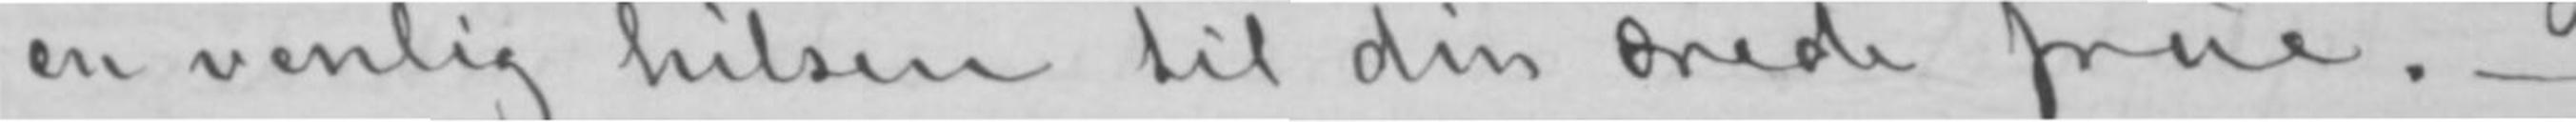en venlig hilsen til din ærede frue.-
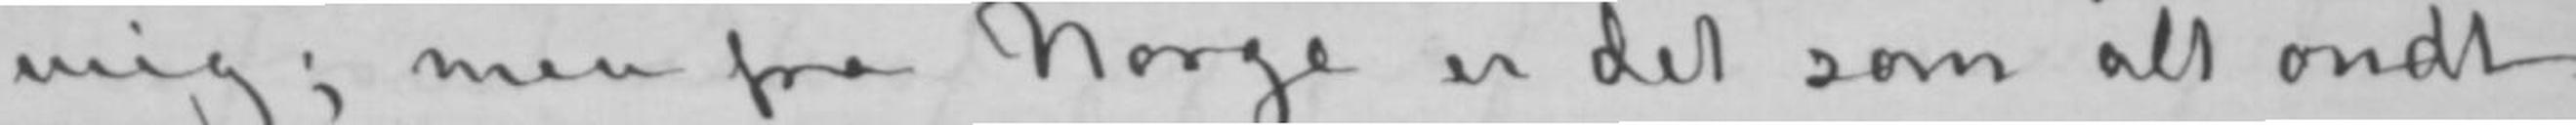mig; men fra Norge er det som alt ondt
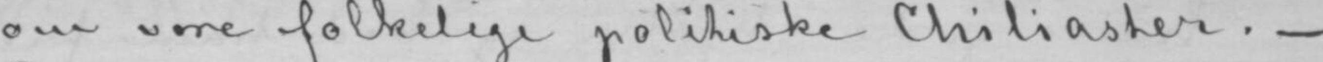om vore folkelige politiske Chiliaster.-
